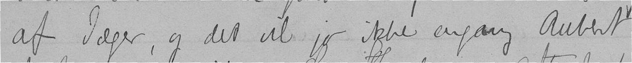af Jæger, og det vil jo ikke engang Aubert
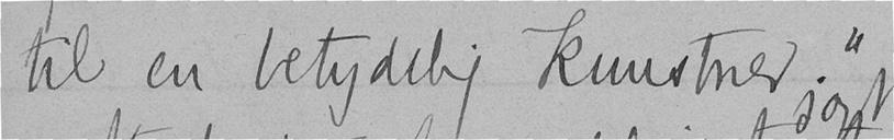til en betydelig Kunstner."
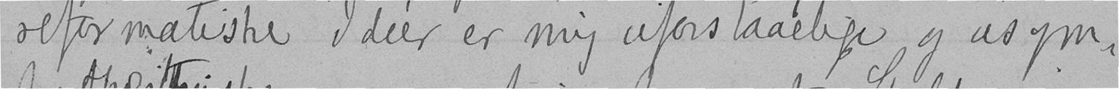reformatiske Idéer er mig uforstaaelige og usym-
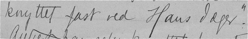knyttet fast ved Hans Jæger".
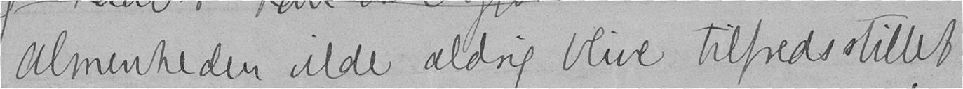Almenheden vilde aldrig blive tilfredsstillet
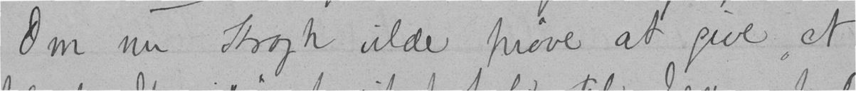Om nu Krogh vilde prøve at give et
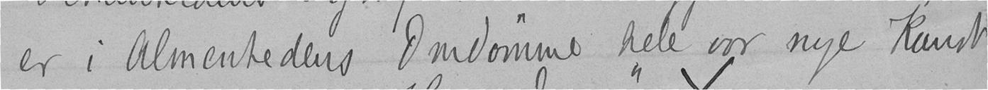er i Almenhedens Omdømme hele vor nye kunst
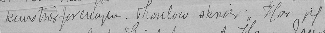kunstnerforeningen. Thaulow skriver "Har jeg
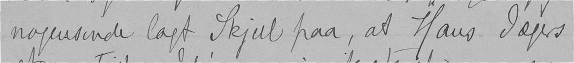nogensinde lagt Skjul paa, at Hans Jægers
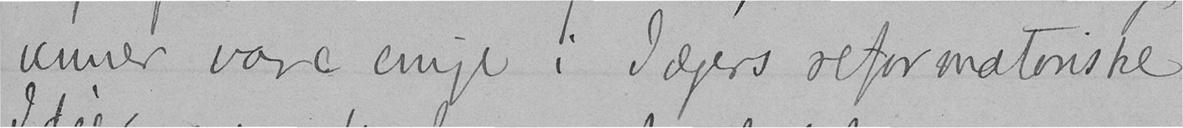venner vare enige i Jægers reformatoriske
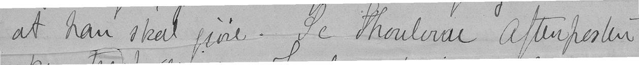at han skal gjøre. Se Thaulowe Aftenposten
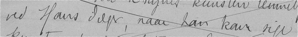ved Hans Jæger, naar han kan sige
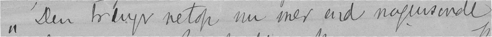"Den trænger netop nu mer end nogensinde
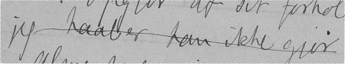jeg haaber han ikke gjør
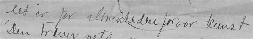Det er for almenheden for vor kunst
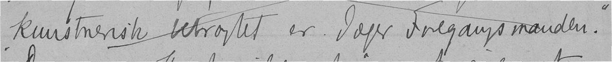"kunstnerisk betragtet er Jæger Foregangsmanden."
